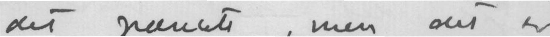det pæneste, men det er
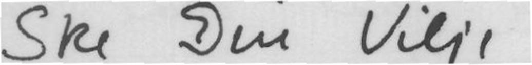Ske Din Vilje.
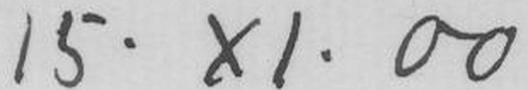15.XI.00
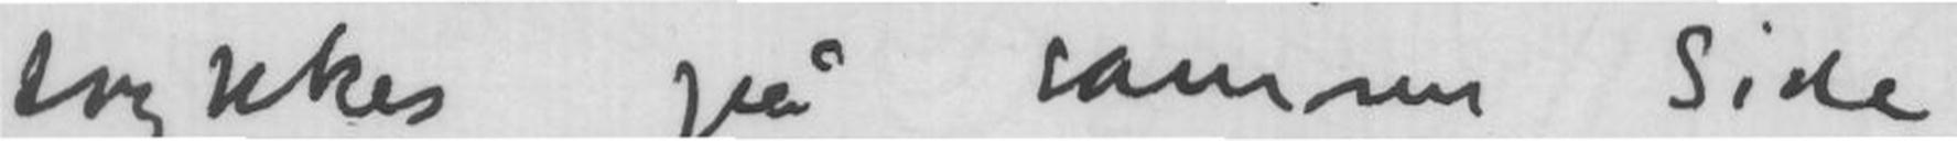trykkes på samme side
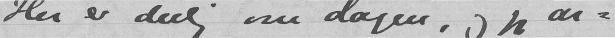Her er deilig om dagen, og jeg ar-
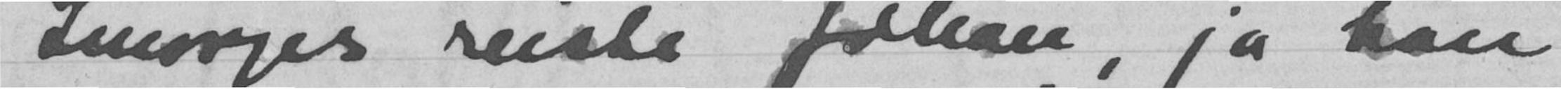Imorges reiste Johan, ja han
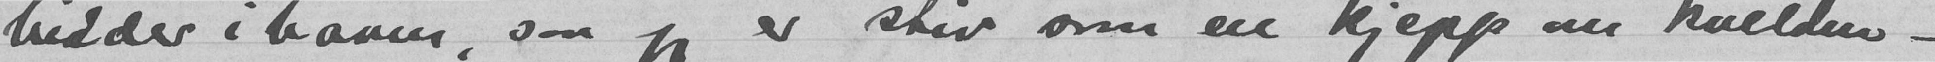beider i haven, saa keh er stiv som en kjepp om kvelden -
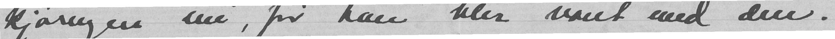kjøringen nu, før han blir vant med den.
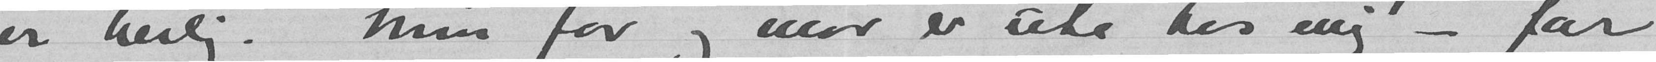er herlig. Min far og mor er ute hos mig - far
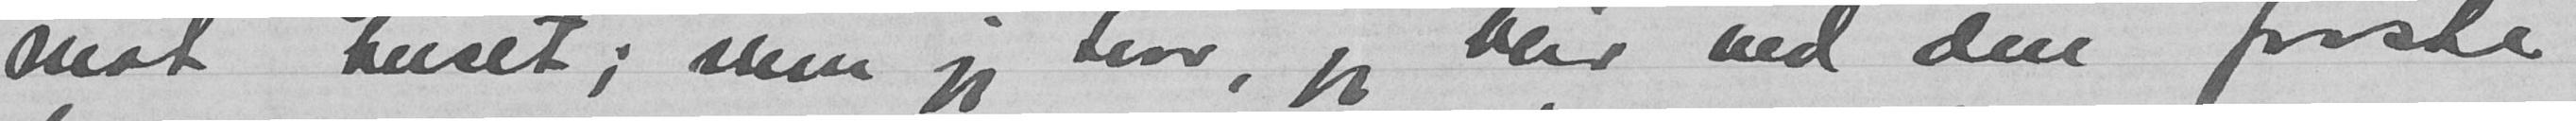mot huset; men jeg tror, jeg blir ved den første
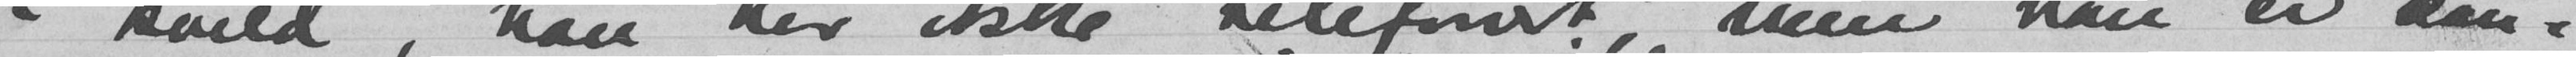i kveld, han har ikke telefonert, men han er kan-
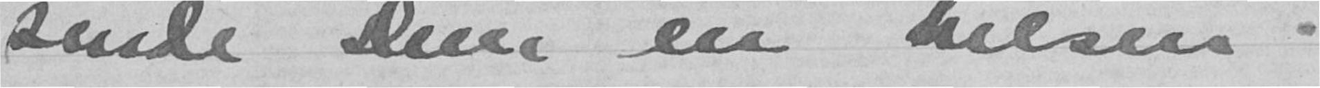sende Dem en hilsen.
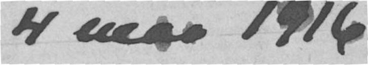4 mai 1916
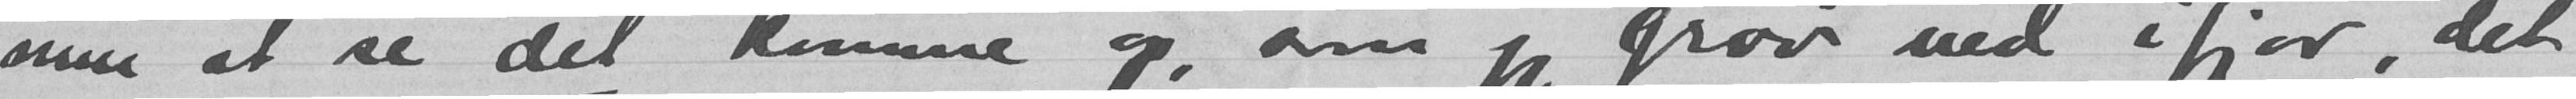men at se det komme op, som jeg grov ned ifjor, det
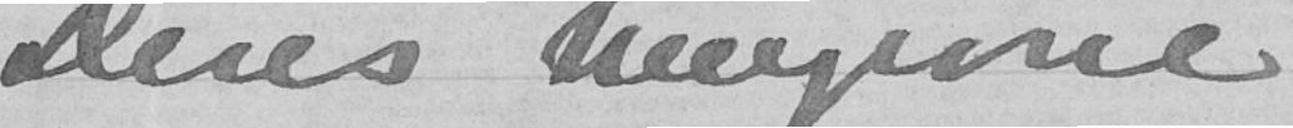Deres hengivne
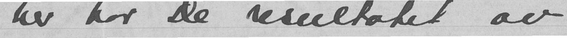Her har De resultatet av
